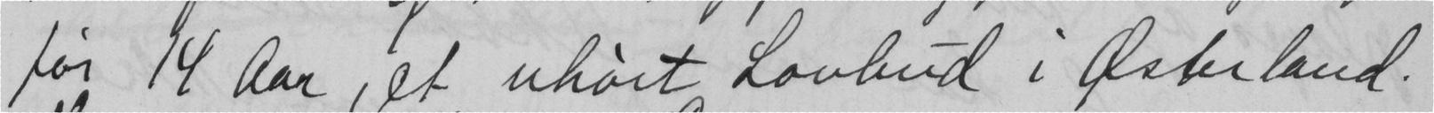før 14 Aar, et uhørt Lovbud i Østerland.
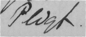Pligt.
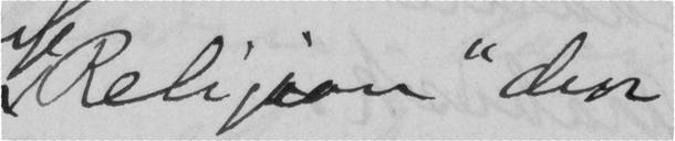Religion "den
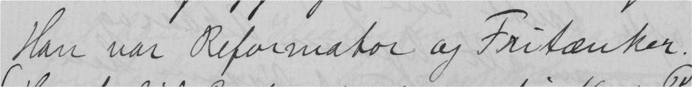Han var Reformator og Fritænker.
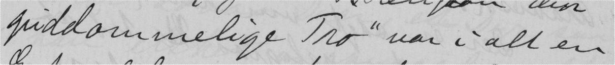guddommelige Tro" var i alt en
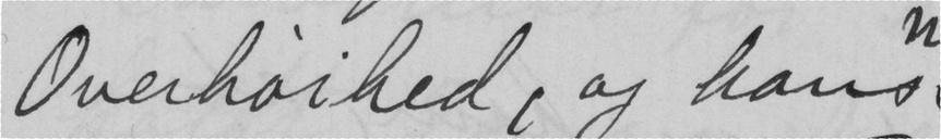Overhøihed, og hans
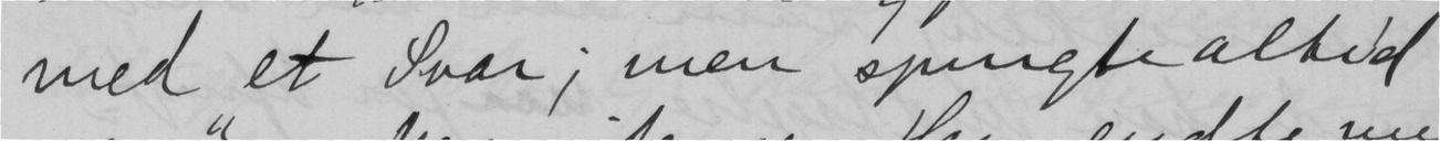med et Svar; men spurgte altid
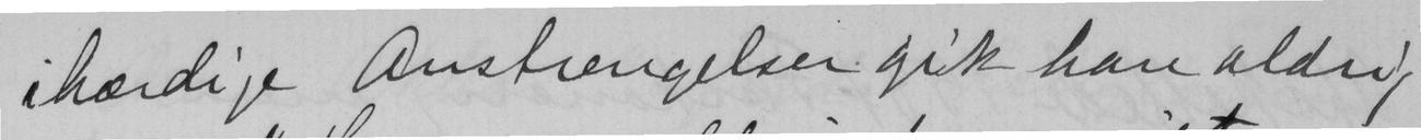ihærdige Anstrengelser gik han aldrig
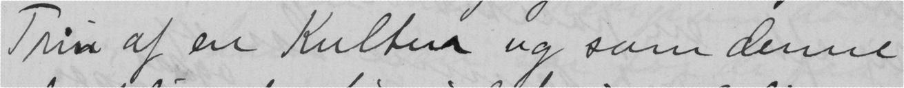Trin af en Kultur ug som denne
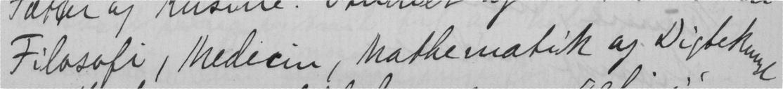Filosofi, Medisin, Mathematik og Digtekunst
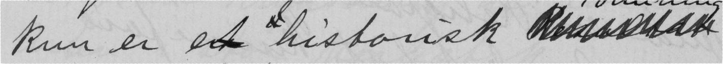kun er et historisk
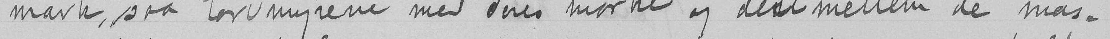mark, saa torvmyrene med deres mørke og derimellem de mas-
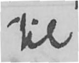til
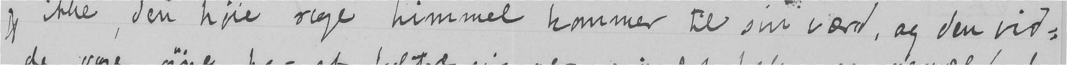jeg ikke, den høie rige himmel kommer til sin værd, og den vid-
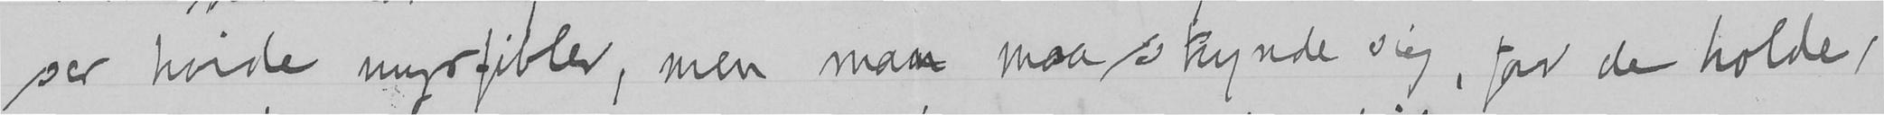ser hvide myrfibler, men man maa skynde sig, for de holde,
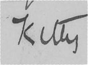Kitty
